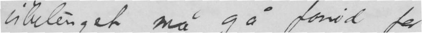ubetinget må gå forud for
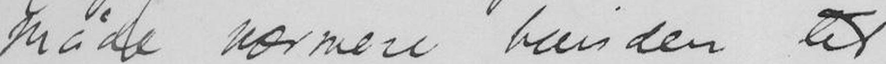måde nærmere bunden til
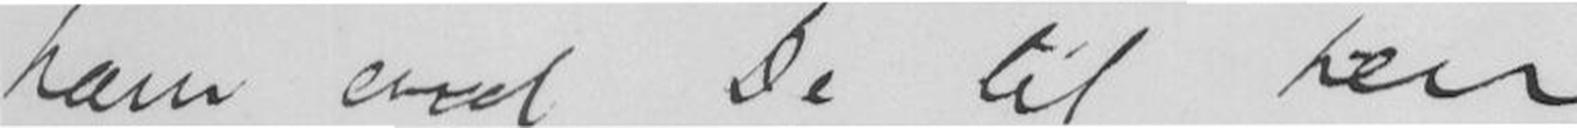ham end De til herr
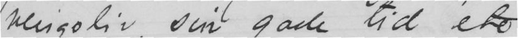ningsliv, sin gode tid etc
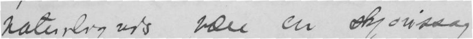naturligvis være en skjønssag.
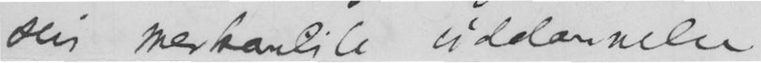sin merkantile uddannelse
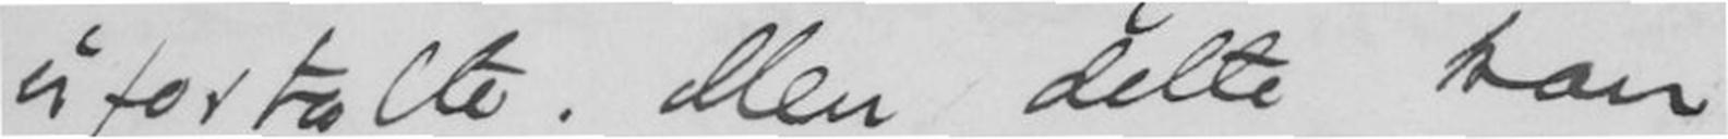ufortalte. Men dette kan
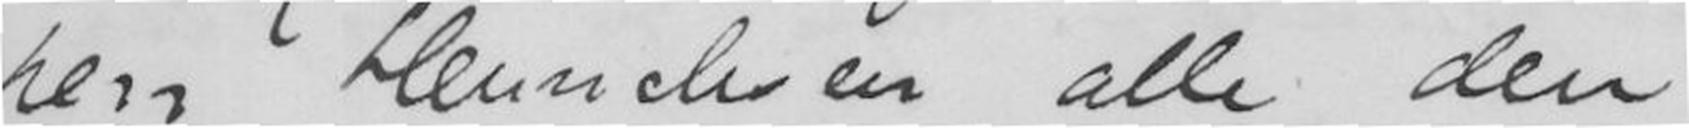herr Henricksen, alle den
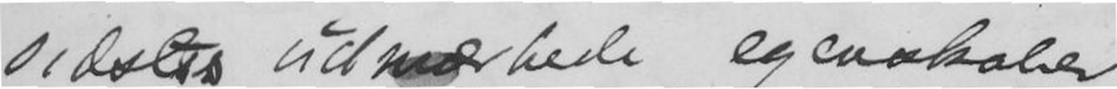sidstes udmærkede egenskaber
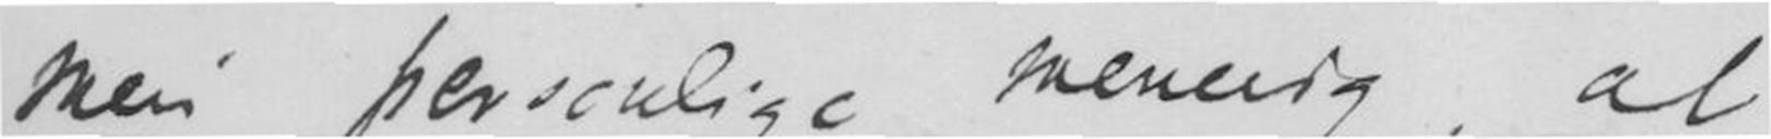min personlige mening, at
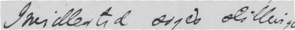Imidlertid søges stillingen
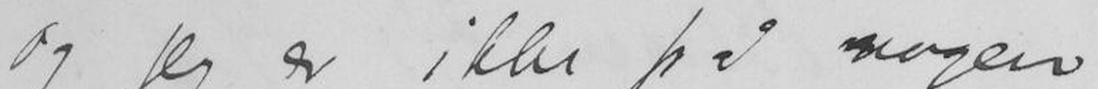og jeg er ikke på nogen
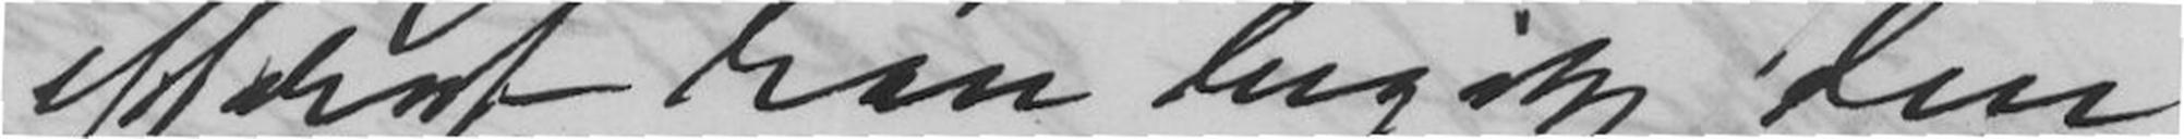efterat han begik den
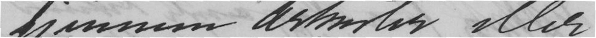gjennem Sang eller
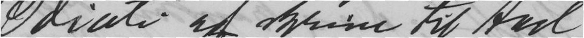Idioti at skrive til Axel
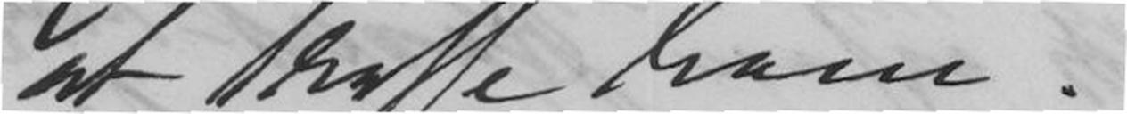at træffe ham. -
